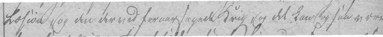Læsion, og den derved foraarsagede Krig, og det kan ogsaa være
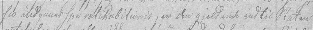hid indgaaes spe ratihabitionis, er den gjeldende indtil Staten
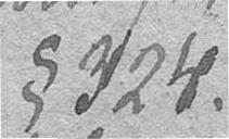§ 324.
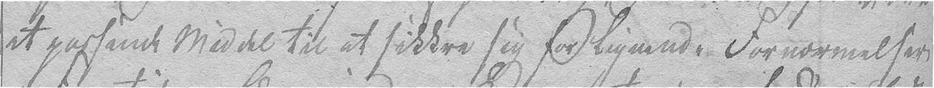et passende Middel til at sikkre sig for lignende Fornærmelser
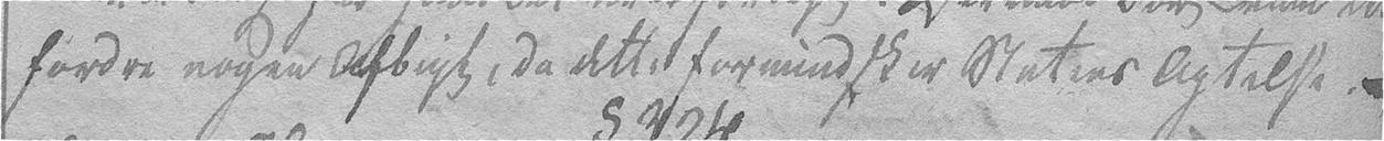fordre nogen Afbigt, da dette formindsker Statens Agtelse. –
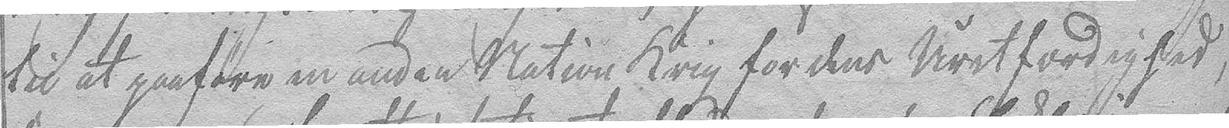til at paaføre en anden Nation Krig for dens Uretfærdighed,
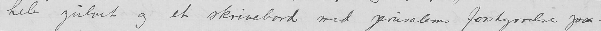hele gulvet og et skrivebord med jerusalems forstyrrelse paa –
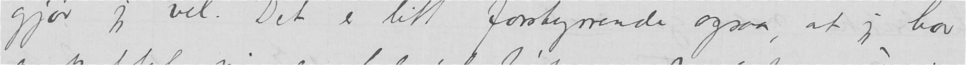gjør jeg vel. Det er litt forstyrrende ogsaa, at jeg har
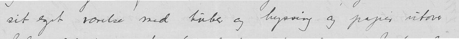sit eget værelse med tuber og hyssing og papir utover
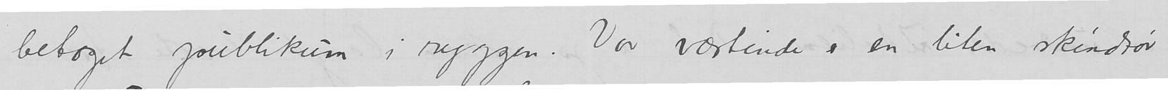betaget publikum i ryggen. Vor værtinde er en liten skindtør
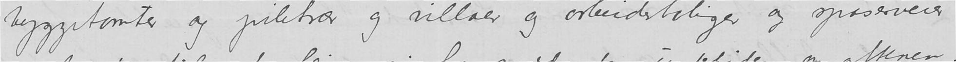byggetomter og juletrær og villaer og arbeiderboliger og spaserveier
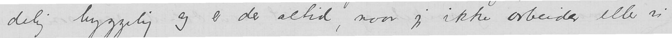delig hyggelig og er der altid, naar jeg ikke arbeider eller
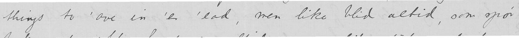things to ‘ave in ‘er ‘ead, men like blid altid, som spør
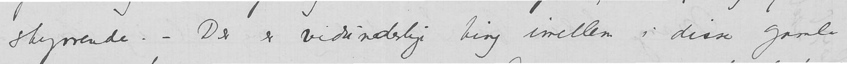styrrende. – –. Der er vidunderlige ting imellem i disse gamle
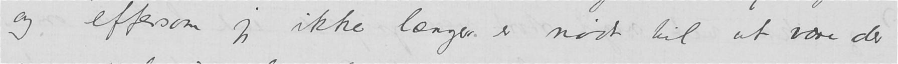og eftersom jeg ikke længer er nødt til at være der
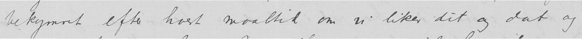bekymret efter hvert maaltid om vi liker dit og dat og
